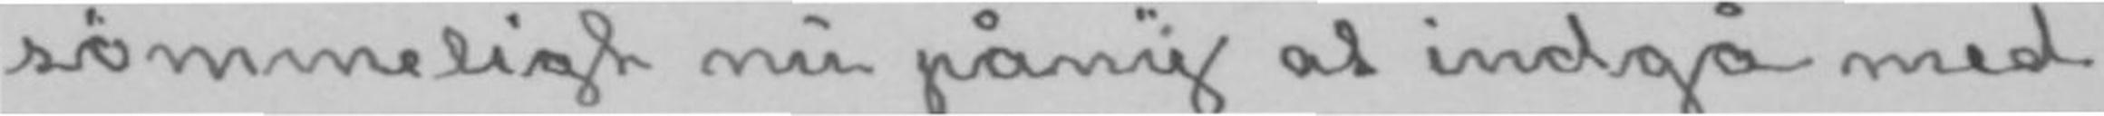sømmeligt nu påny at indgå med
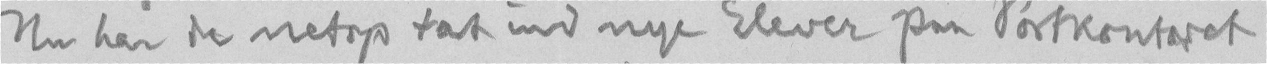Nu har de netop tat ind nye Elever paa Postkontoret
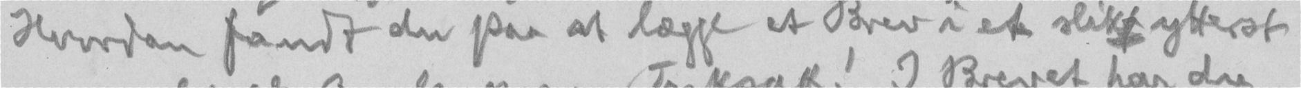Hvordan fandt du paa at lægge et Brev i et slikt ytterst
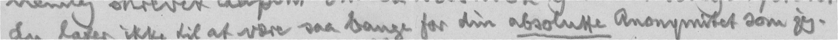du later ikke til at være saa bange for din absolutte Anonymitet som jeg.
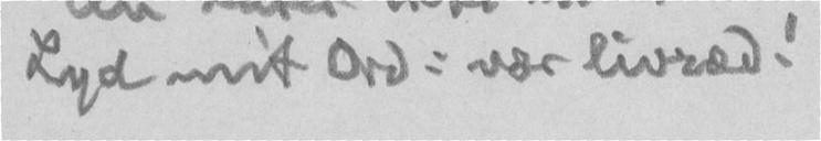Lyd mit Ord: vær livræd!
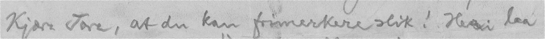Kjære Tore, at du kan frimerkere slik! Heri laa
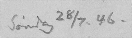Søndag 28/7. 46.
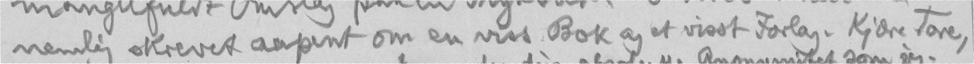nemlig skrevet aapent om en viss Bok og et visst Forlag. Kjære Tore,
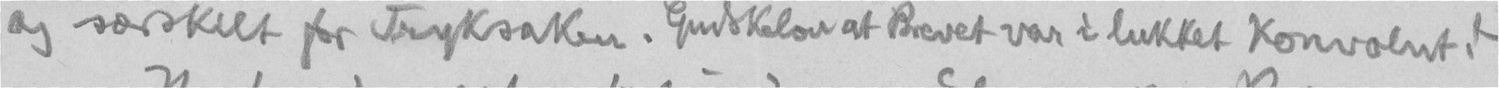og særskilt for Tryksaken. Gudskelov at Brevet var i lukket Konvolut!
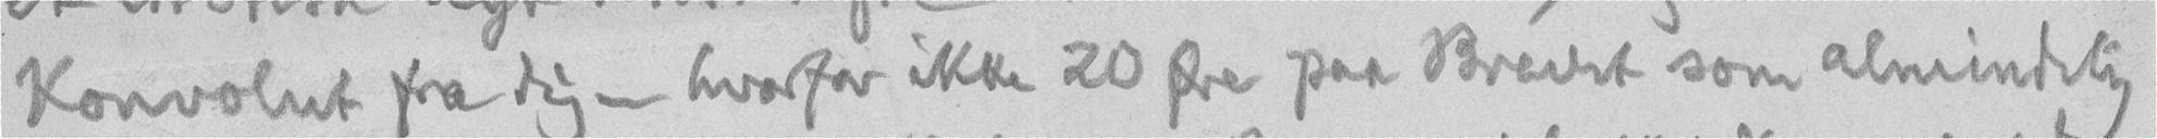Konvolut fra dig - hvorfor ikke 20 Øre paa Brevet som almindelig
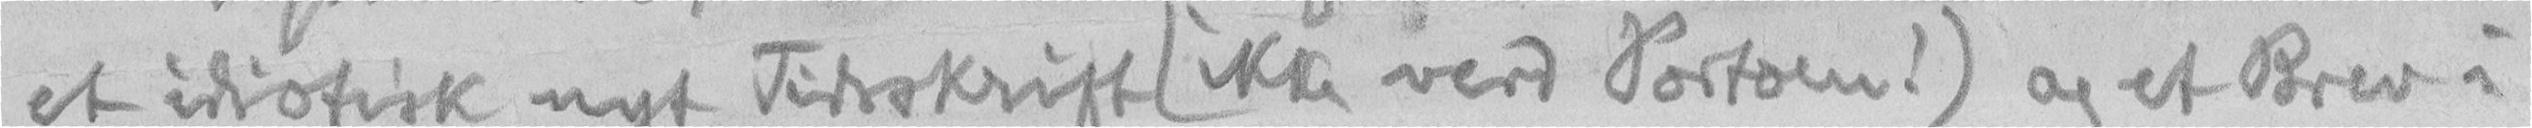et idiotisk nyt Tidsskrift (ikke verd Portoen!) og et Brev i
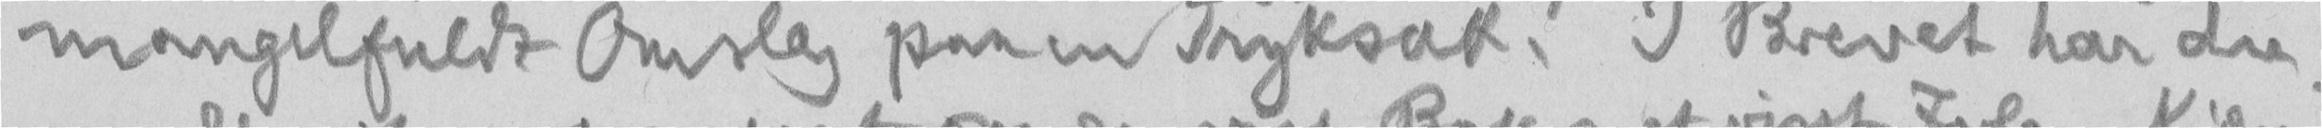mangelfuldt Omslag paa en Tryksak! I Brevet har du
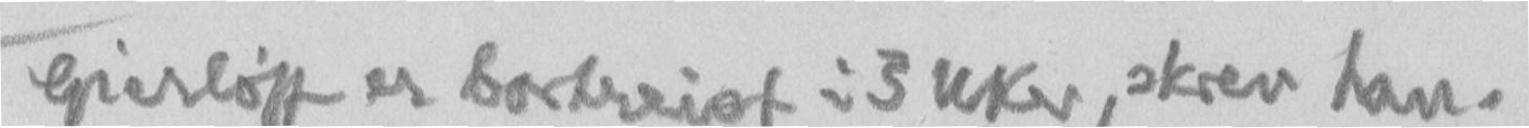Gierløff er bortreist i 3 uker, skrev han.
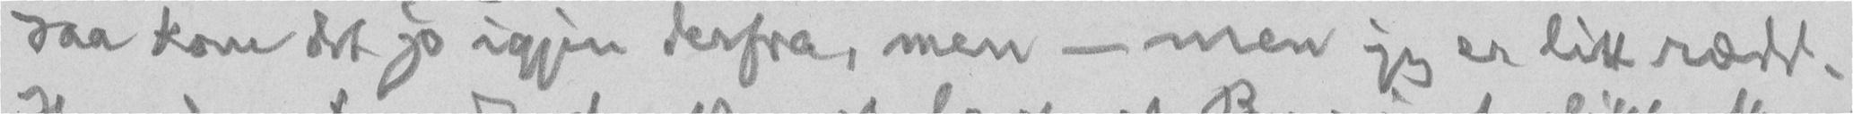saa kom det jo igjen derfra, men - men jeg er litt rædd.
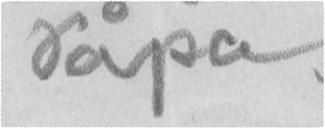Papa
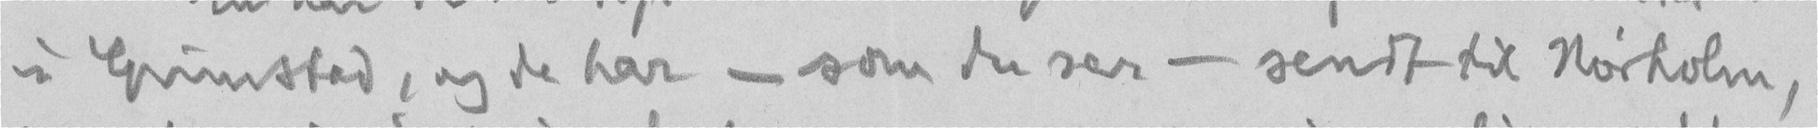i Grimstad, og de har - som du ser - sendt til Nørholm,
